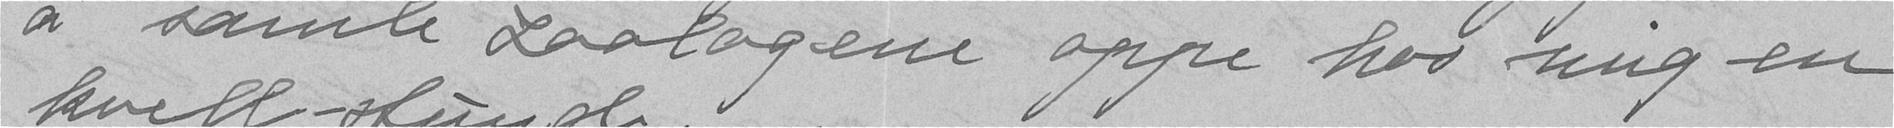å samle zoologene oppe hos nng en
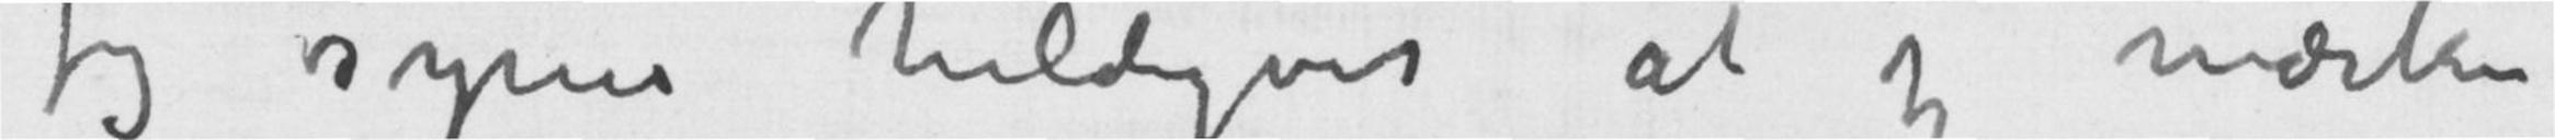Jeg synes heldigvis at jeg mærker
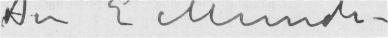Din E Munch –
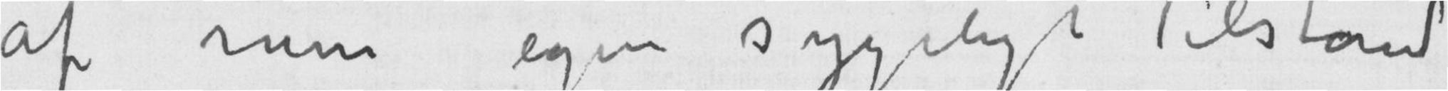af min egen sygelige Tilstand
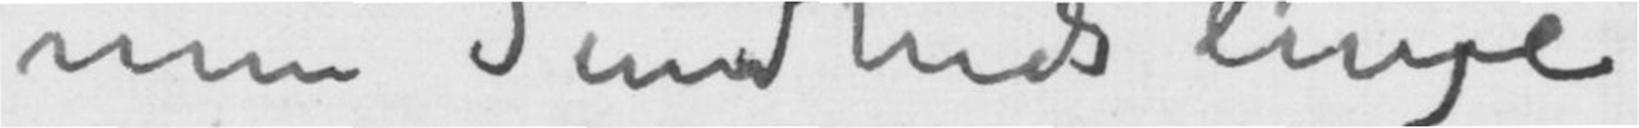i min Sundhedslinje
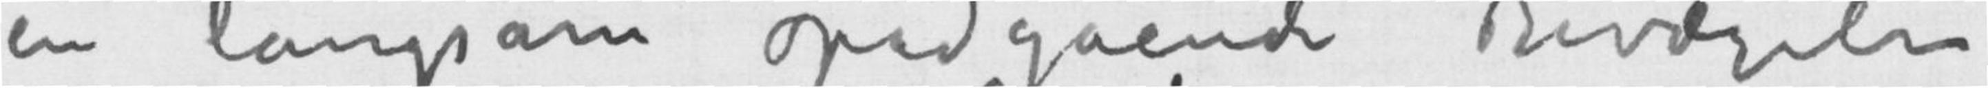en langsom opadgående Bevægelse
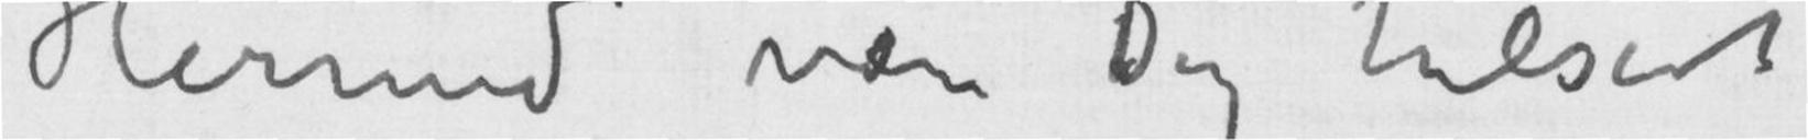hermed være Dig hilset
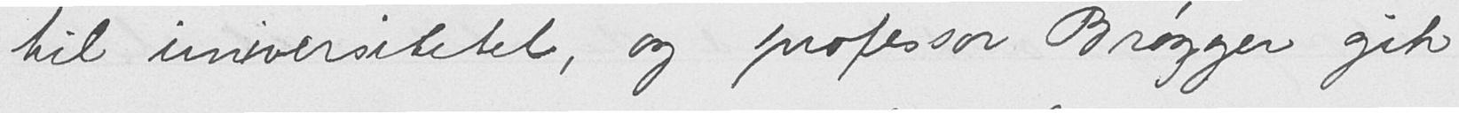til universitetet, og professor Brøgger gik
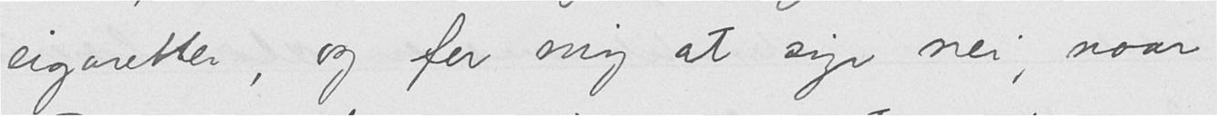cigaretter, og for mig at sige nei, naar
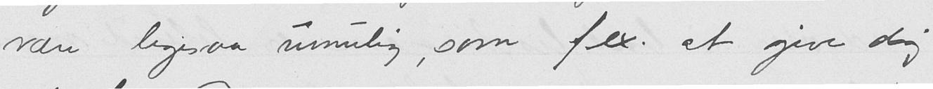være ligesaa umulig, som f ex. at give dig
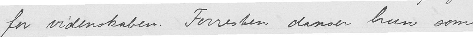for videnskaben. Forresten danser hun som
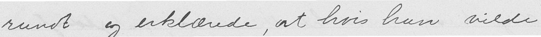rundt og erklærede, at hvis hun vilde
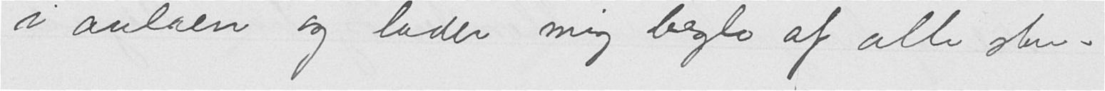i aulaen og lader mig beglo af alle stu-
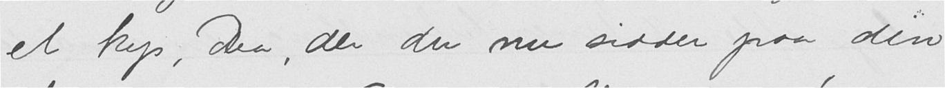et kys, Dea, der du nu sidder paa din
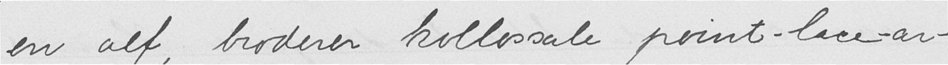en alf, broderer kollossale point-lace-ar-
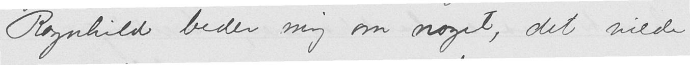Ragnhild beder mig om noget, det vilde
